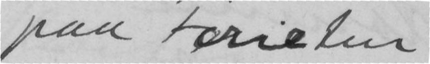paa Forreten
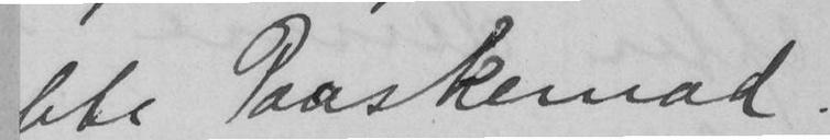bti Paaskemad
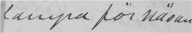Campa før nasan
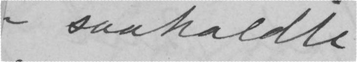- saakaldte
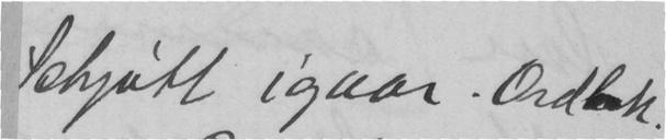Schjøtt igaar. Ordbok.
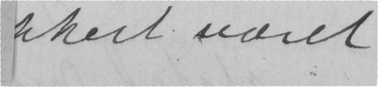kkert været
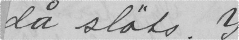da sløte?
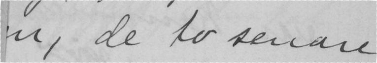m; de to senare
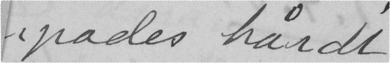grades har dt
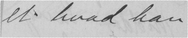et hvad han
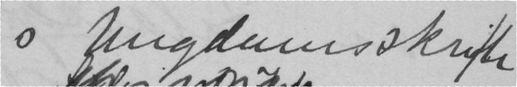s Ungdomsskrifte
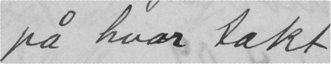på hvar takt.
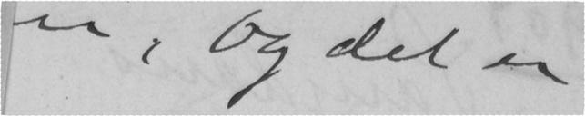er. Og det er
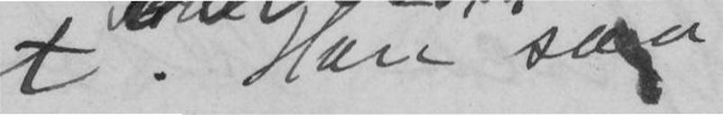t. Han saa
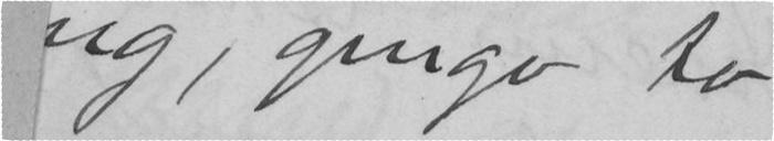sg, gingv to
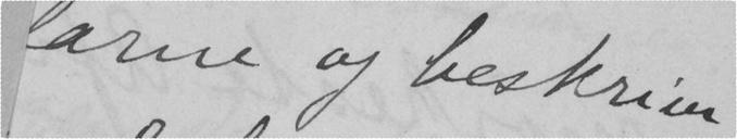arne og beskrive
